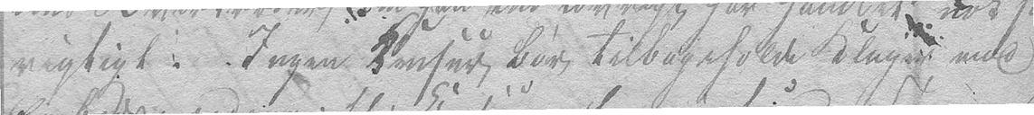rigtigt. Ingen Censur bør tilbageholde Klager mod
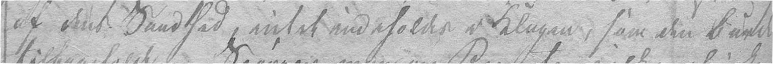af dens Sandhed, intet indeholdes i Klagen, som den burde
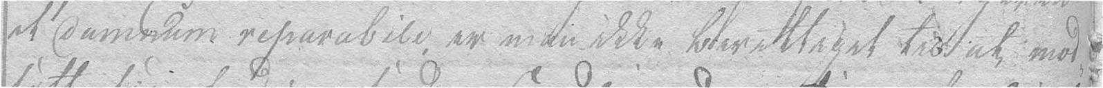et damnum reparabile er man ikke berettiget til at mod-
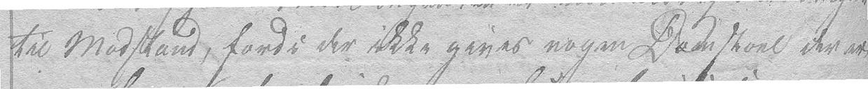til Modstand, fordi der ikke gives nogen Domstoel der er
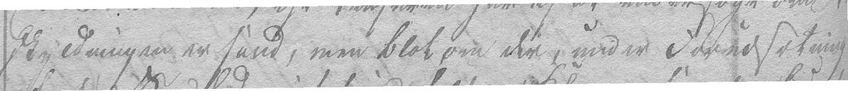Skyldningen er sand, men blot om der, under Forudsætning
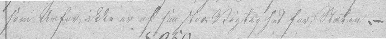som derfor ikke er af saa stor Vigtighed for Staten. –
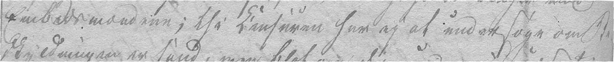Embedsmændene; thi Censuren har ey at undersøge om
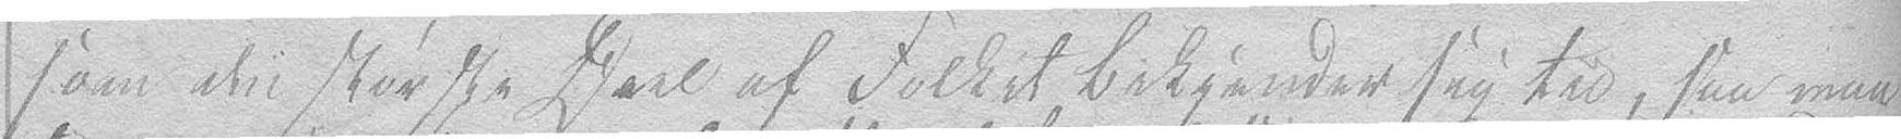som den største Deel af Folket bekjender sig til, saa maa
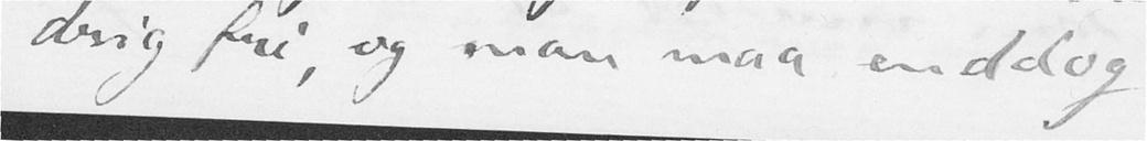drig fri, og man maa enddog
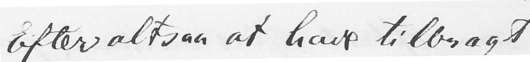Efter altsaa at have tilbragt
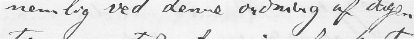nemlig ved denne ordning af dagen
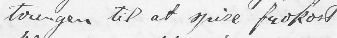tvungen til at spise frokost
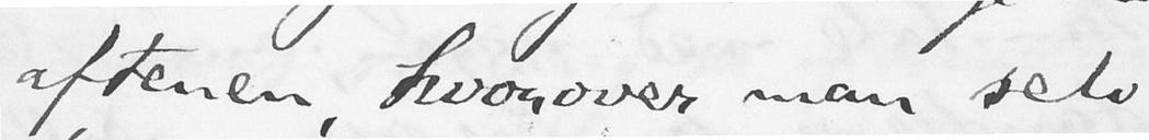aftenen, hvorover man selv
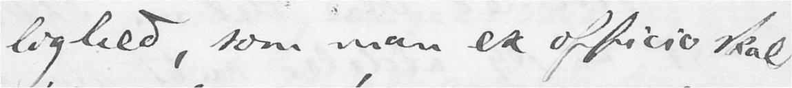lighed, som man ex officio skal
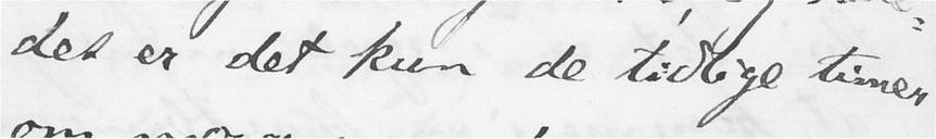des er det kun de tidlige timer
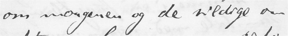om morgenen og de sildige om
